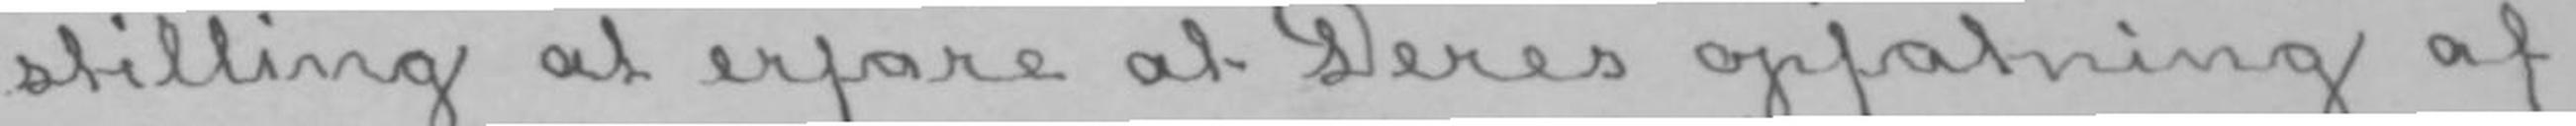stilling at erfare at Deres opfatning af
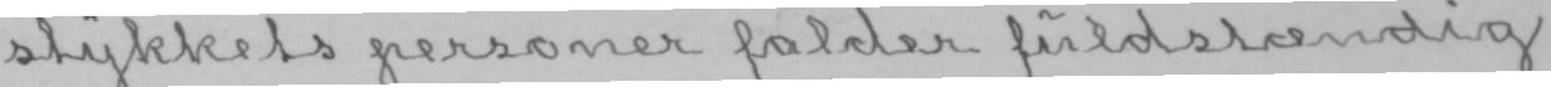stykkets personer falder fuldstændig
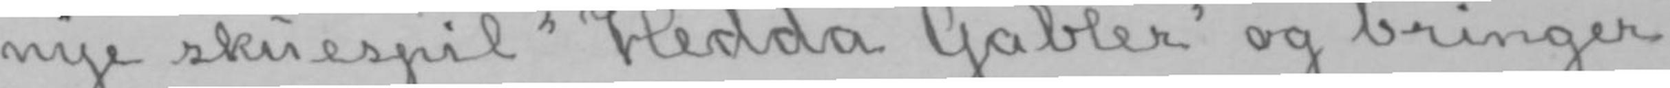nye skuespil «Hedda Gabler» og bringer
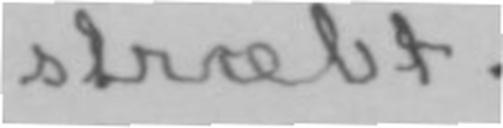stræbt.
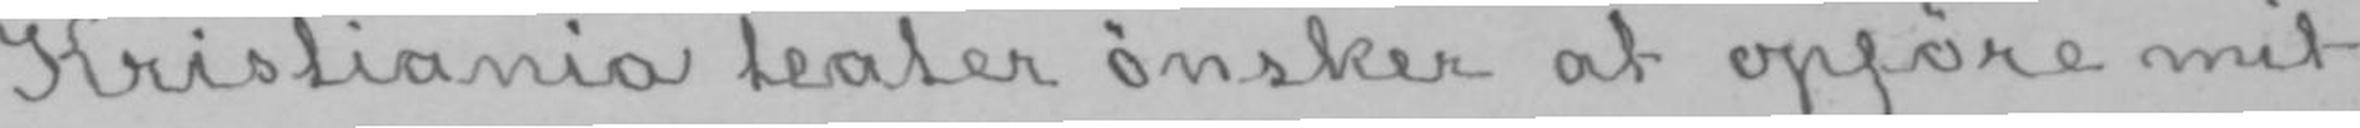Kristiania teater ønsker at opføre mit
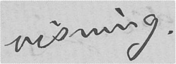visning.
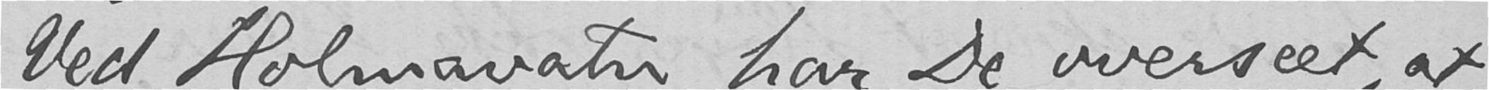Ved Holmavatn har De overseet, at
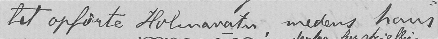tet opførte Holmavatn, medens hans
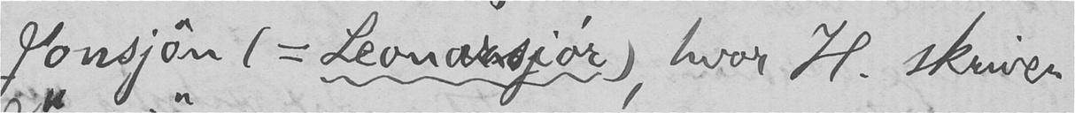Jonsjön (= Leonarsjór), hvor H. skriver
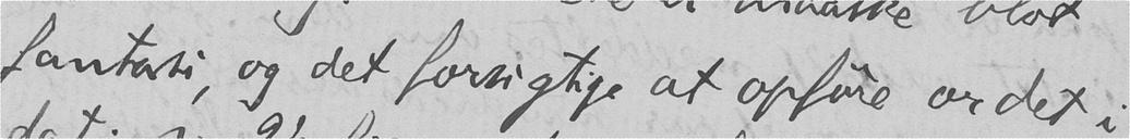fantasi, og det forsigtige at opføre ordet i
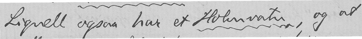Lignell ogsaa har et Holmvatn, og at
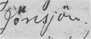Jönsjön.
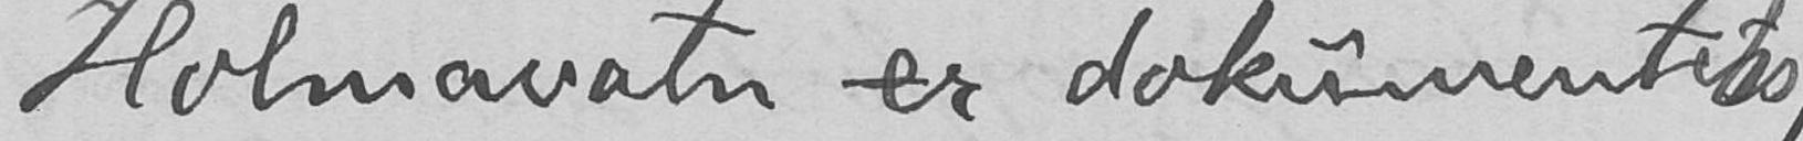Holmavatn er dokumentets
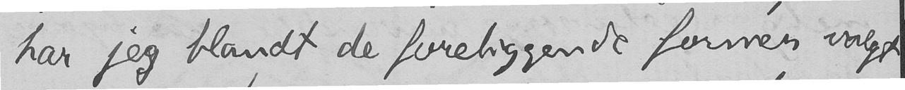har jeg blant de foreliggende former valgt
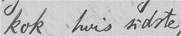kok, hvis sidste
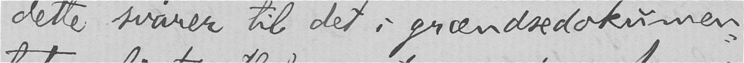dette svarer til det i grændsedokumen-
